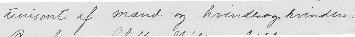unisont af mænd og kvindesagskvinder.
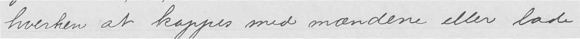hverken at kappes med mændene eller lade
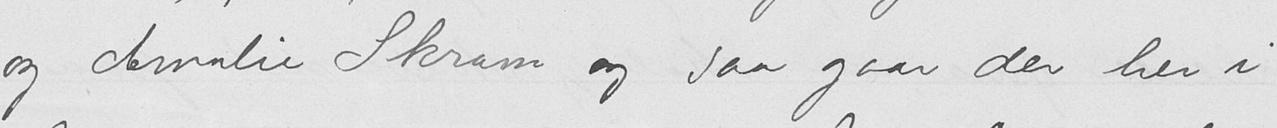og Amalie Skram og saa gaar der her i
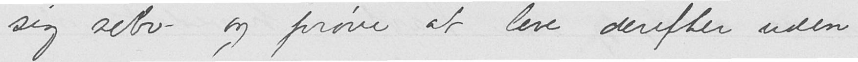sig selv og prøve at leve derefter uden
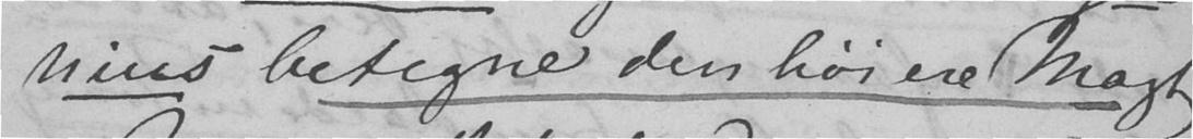nius betegne den høiere Magt
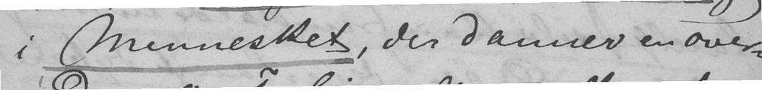i Mennesket, der danner en over-
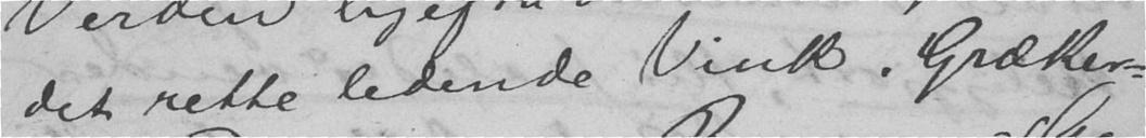det rette ledende Vink. Græker-
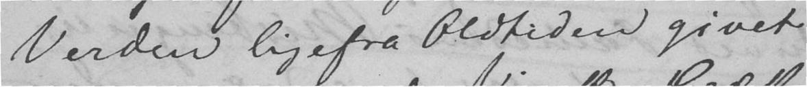Verden ligefra Oldtiden givet
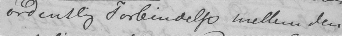ordentlig Forbindelse mellem den
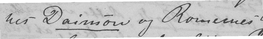nes Daimon og Romernes Ge-
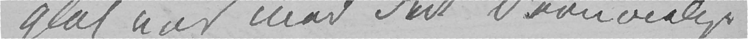glat ned med «det Fornøielige»
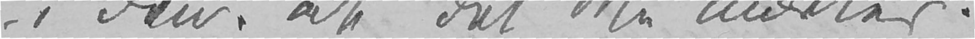i den, at det ikke mærkes.
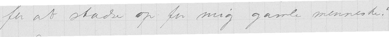for at stadse op for mig gamle menneske."
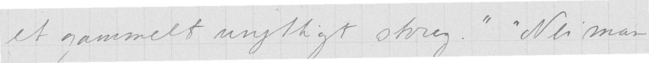et gammelt unyttigt skrog." "Nei man
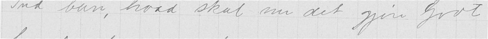Gud børn, hvad skal nu det gjøre godt
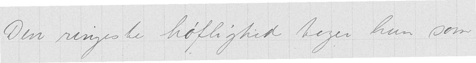Den ringeste høflighed tager hun som
